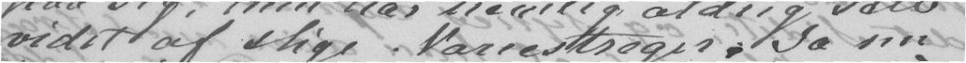vidst af slige Narrestreger. Ja nu
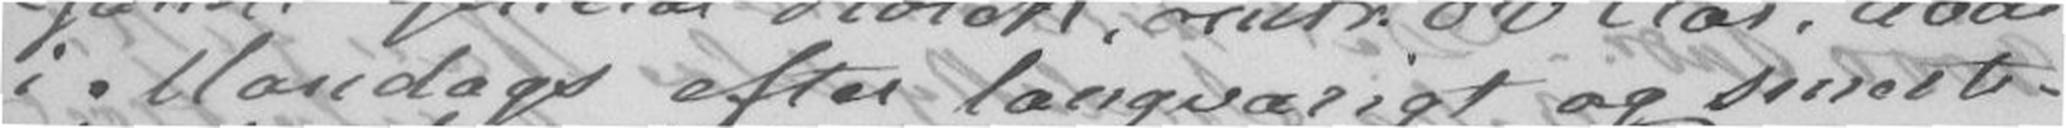i Mandags efter langvarigt og smerte-
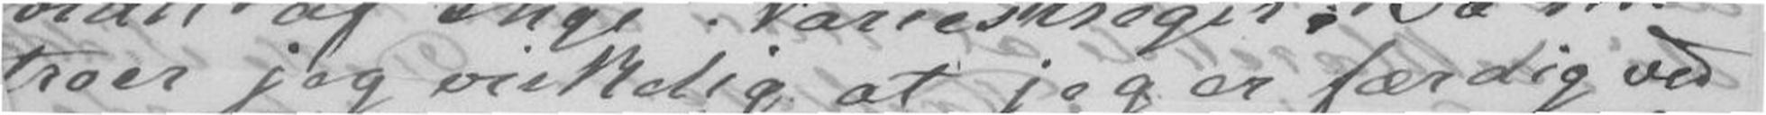troer jeg virkelig at jeg er færdig ved
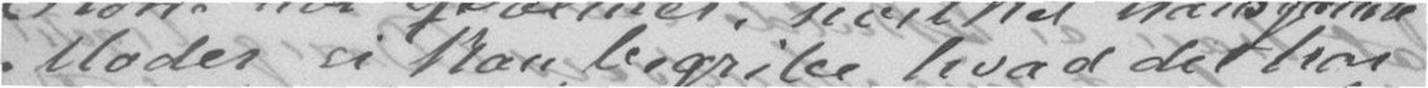Moder ei kan begribe hvad det har
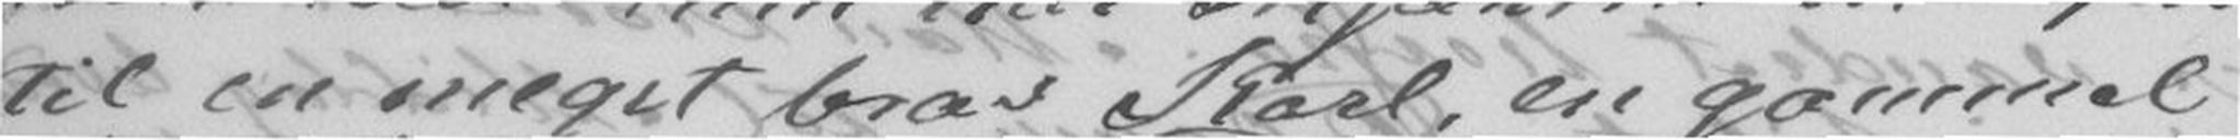til en meget brav Karl, en gammel
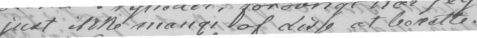just ikke mange af disse at berette.
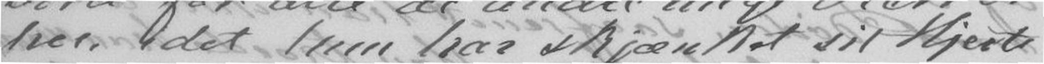her, det hun har skjænket sit Hjerte
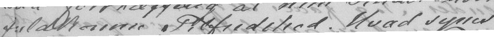fuldkomne Tilfredshed. Hvad synes
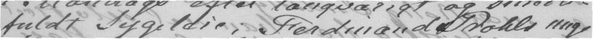fuldt Sygeleie; Ferdinand Prohls unge
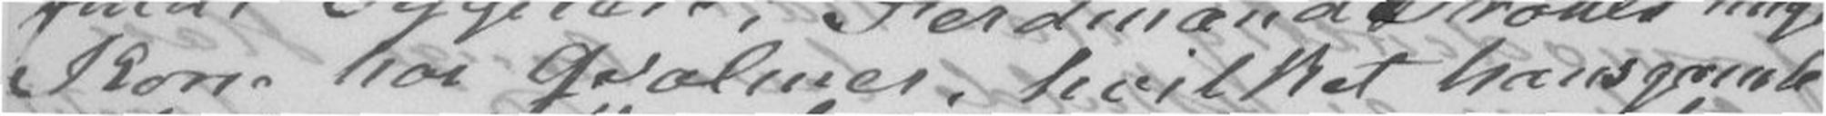Kone har Qvalmer, hvilket hans gamle
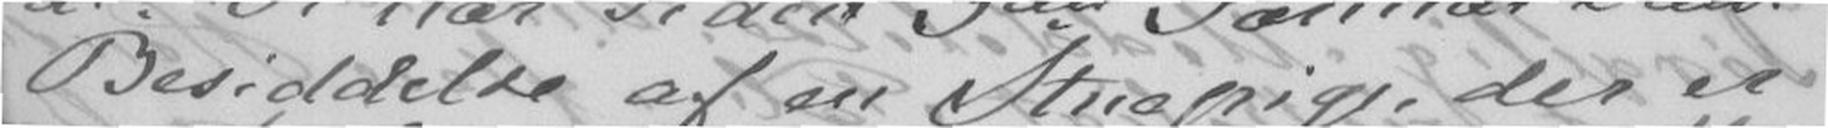i Besiddelse af en Stuepige, der er
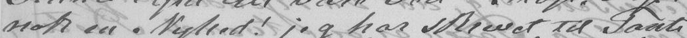nok en Nyhed! jeg har skrevet til Tante
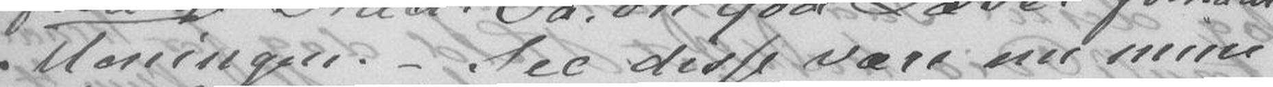Meningen. - See disse være nu mine
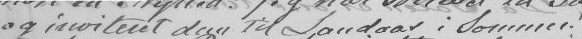og inviteret dem til Landaas i Sommer!
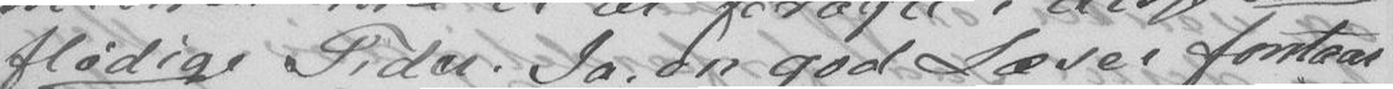flødige Tider. Ja, en god Læser forstaar
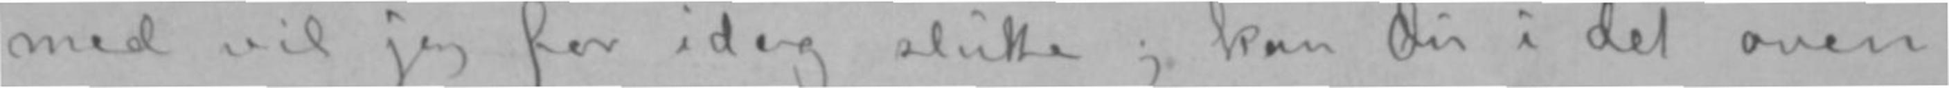med vil jeg for idag slutte; kan Du i det oven-
